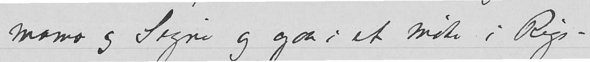momo og Signe og gaa i et møte i Rigs-
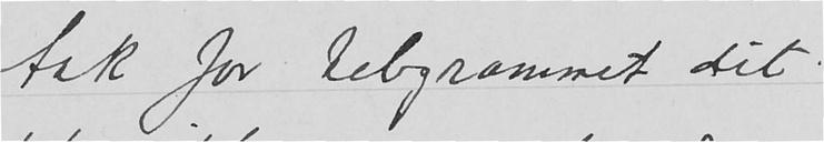tak for telegrammet dit.
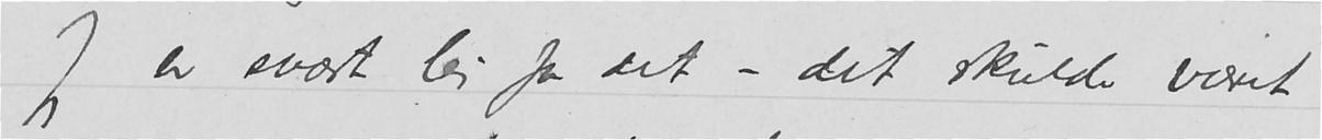Jeg er svært lei for det – det skulde været
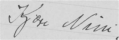Kjære Nini,
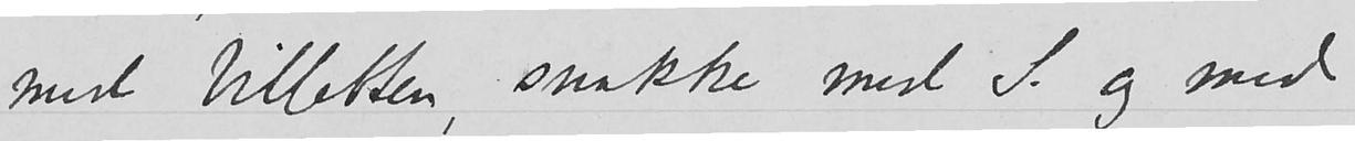med billetten, snakke med S. og med
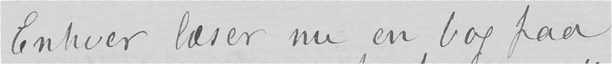Enhver læser nu en bog paa
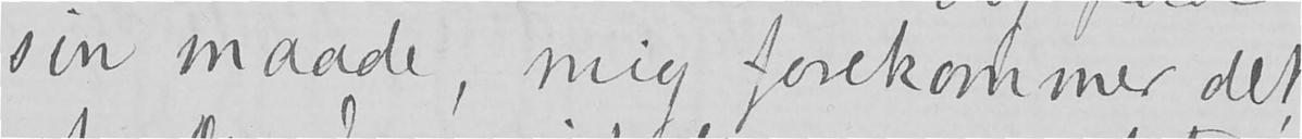sin maade, mig forekommer det,
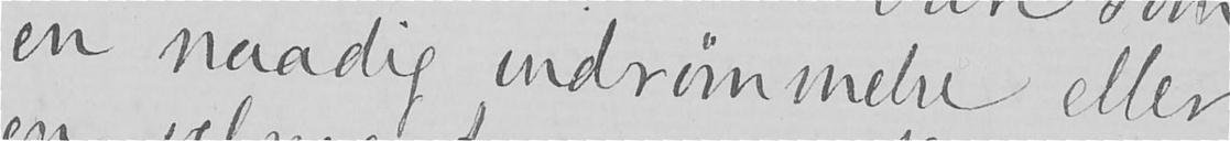en naadig indrømmelse eller
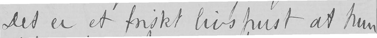Det er et friskt livspust, at hun
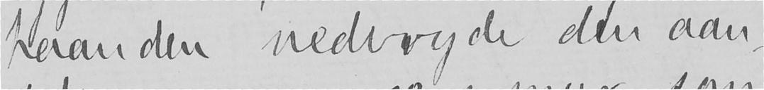haanden nedbryde den aan-
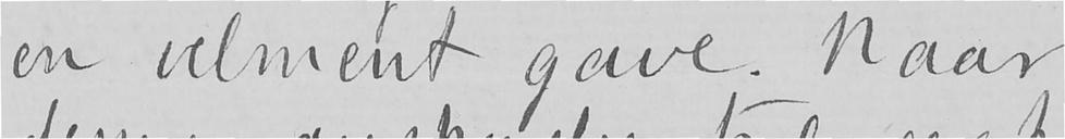en velment gave. Naar
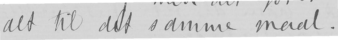alt til det samme maal.
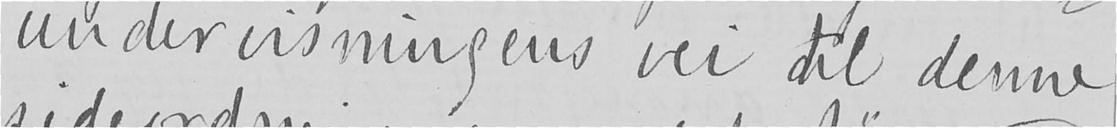undervisningens vei til denne
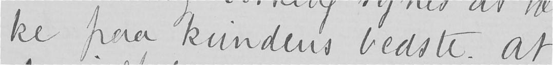ke paa kvindens bedste, at
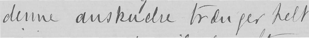denne anskuelse trænger helt
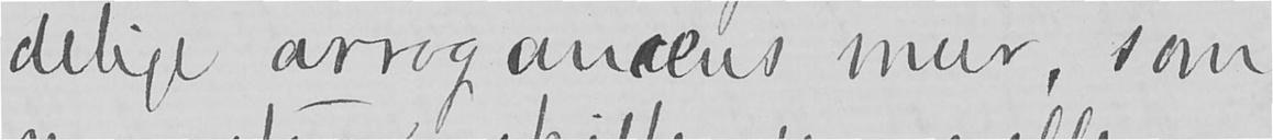delige arrogancens mur, som
